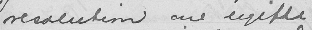resolution om ugifte
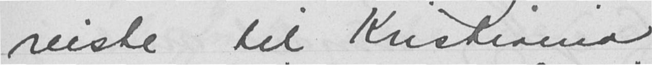reiste til Kristiania
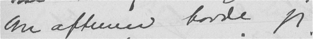Om aftenen havde jeg
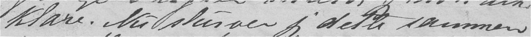klare. Nu slurver jeg dette sammen
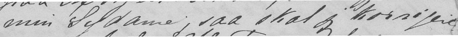min Sydame; saa skal jeg korrigere
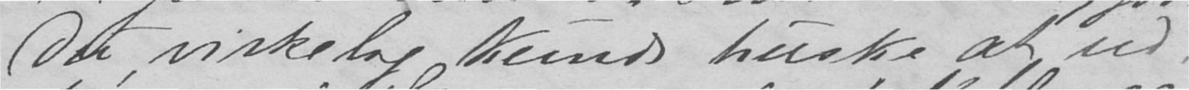Du, virkelig kunde huske at ud-
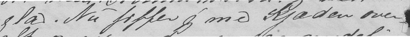glad. Nu fiffer jeg med Kjæden over-
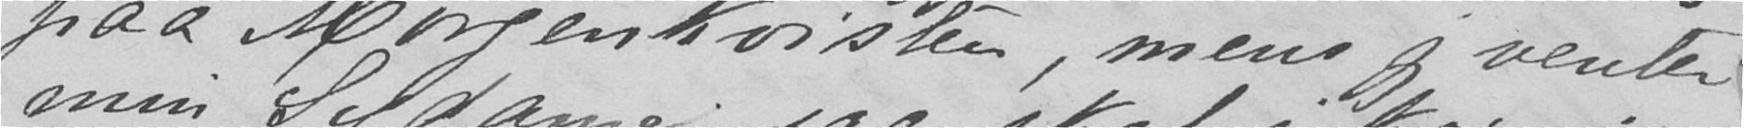paa Morgenkvisten, mens jeg venter
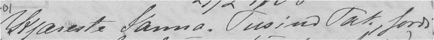Kjæreste Janna. Tusind Tak, fordi
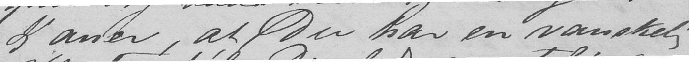Jeg aner, at Du har en vanskelig
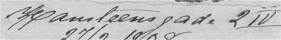Hansteensgade 2 IV
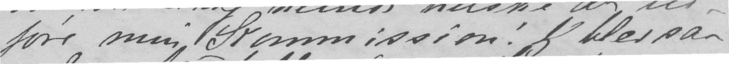føre min Kommission! Jeg blev saa
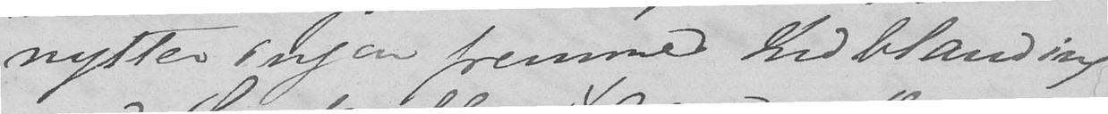nytter ingen fremmed Indblanding
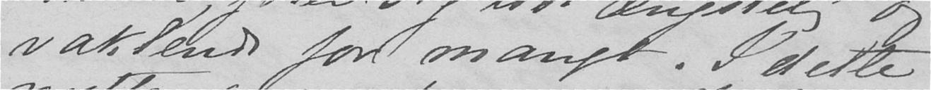vaklende for mangt. I dette
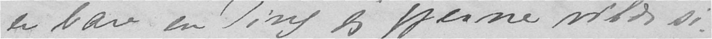er bare en Ting jeg gjerne vilde si.
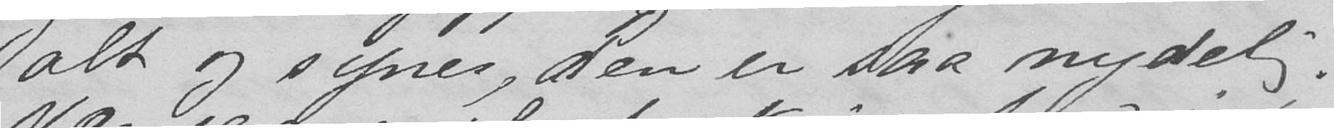alt og synes, den er saa nydelig.
